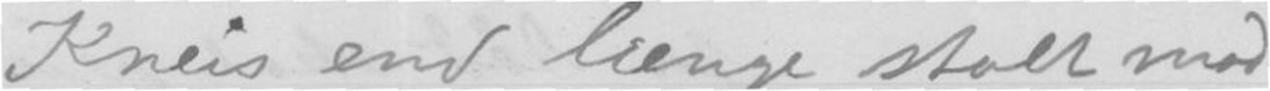Kneis end længe stolt mod
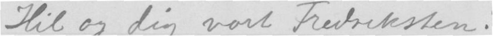Hil og dig vort Fredriksten!
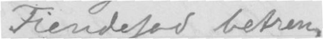Fiendefod betren.
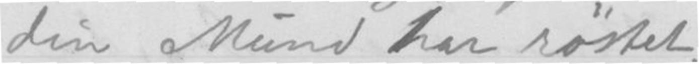din Mund har röstet,
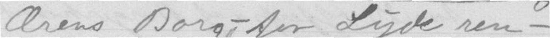Ærens Borg- for Lyde ren -
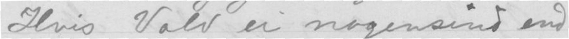Hvis Vold ei Nogensind end
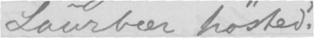Laurbær høsted'.
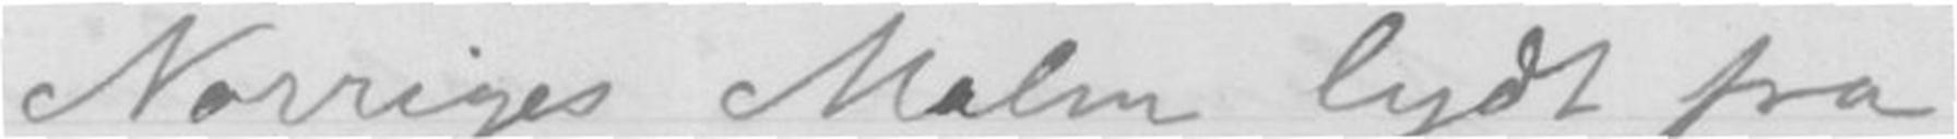Norriges Malm lydt fra
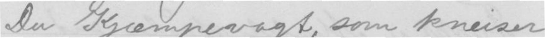Du Kjæmpevagt som kneiser
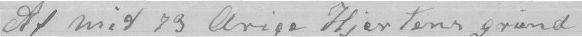Af mit 73 Arige Hjertens grund
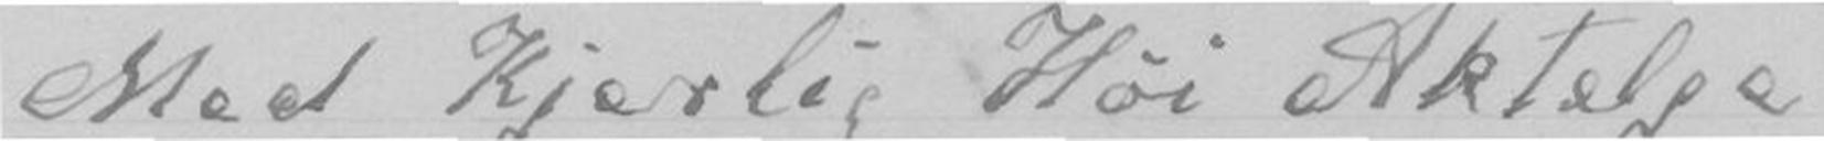Med Kjerlig Høi Agtelse
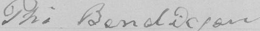Phi. Bendixson
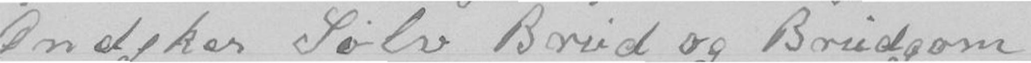Øndsker Sølv Brud og Brudgom
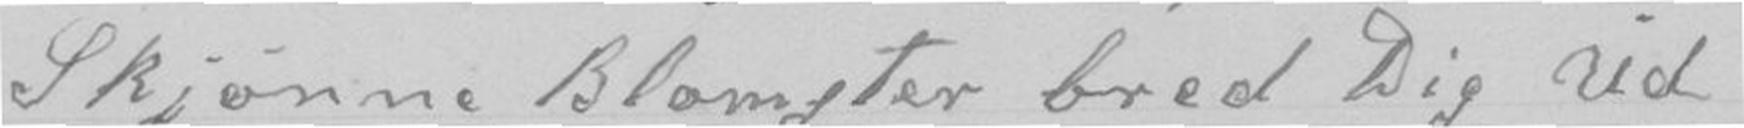Skjønne Blomster bred Dig ud
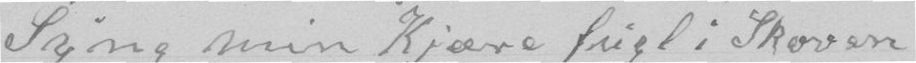Syng min Kjære fugl i Skoven
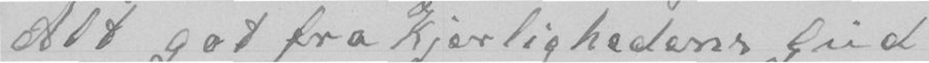Alt got fra Kjerlighedens Gud
